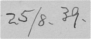25/8. 39.
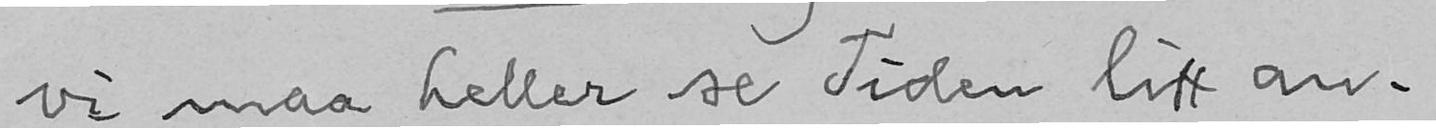vi maa heller se Tiden litt an.
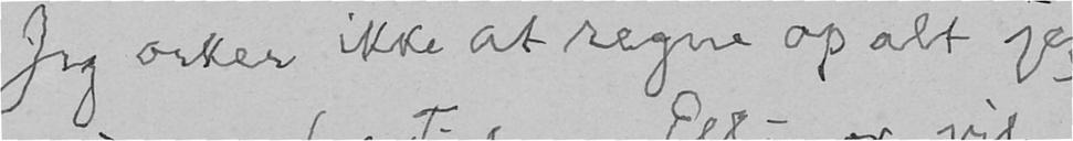Jeg orker ikke at regne op alt jeg
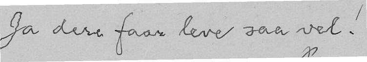Ja dere faar leve saa vel!
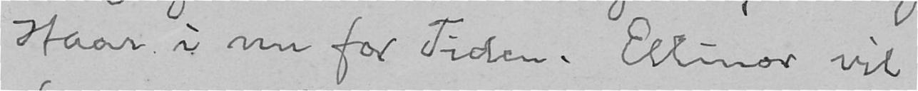staar i nu for Tiden. Ellinor vil
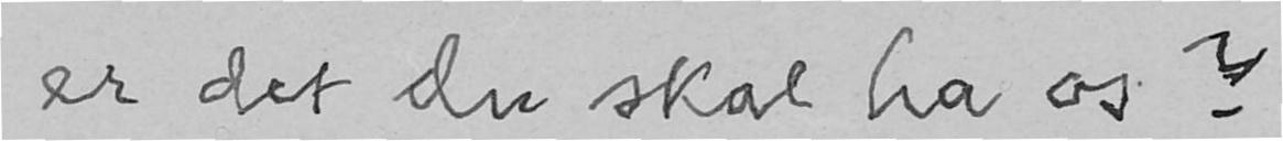er det du skal ha os?
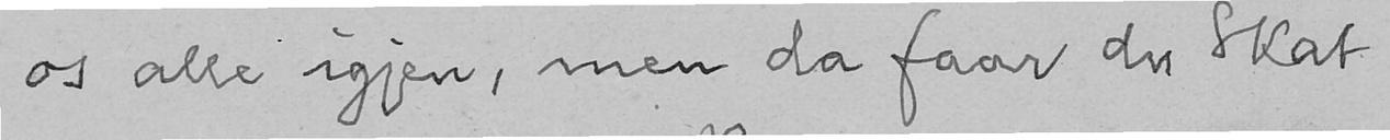os alle igjen, men da faar du Skat
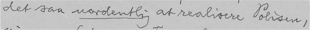det saa uordentlig at realisere Polisen,
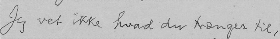Jeg vet ikke hvad du trænger til,
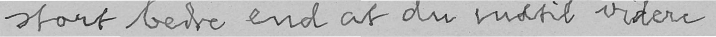stort bedre end at du indtil videre
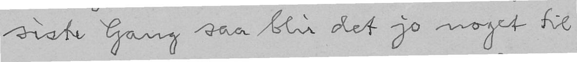siste Gang saa blir det jo noget til
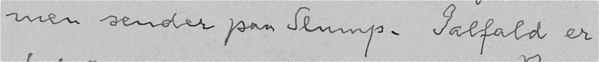men sender paa Slump. Ialfald er
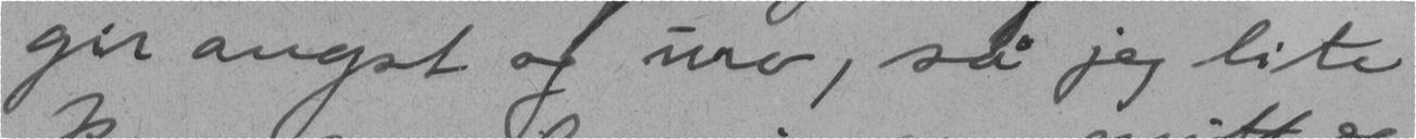gir angst og uro, så jeg lite
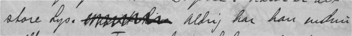store lys.  Aldrig har han endnu
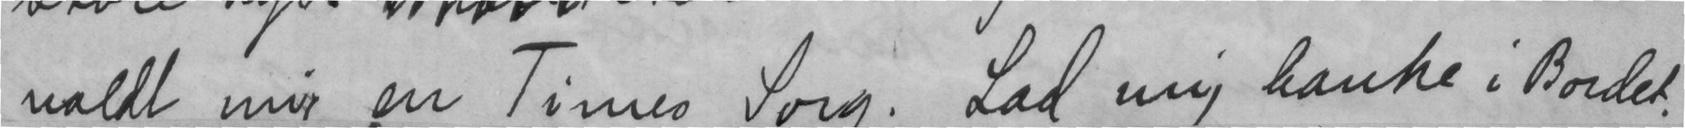voldt mig en Times Sorg. Lad mig banke i Bordet.
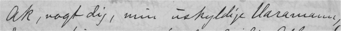Ak, vogt dig, min uskyldige varamann,
